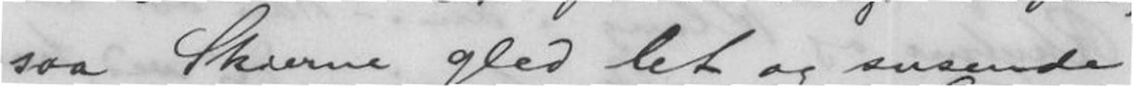saa Skierne gled let og susende
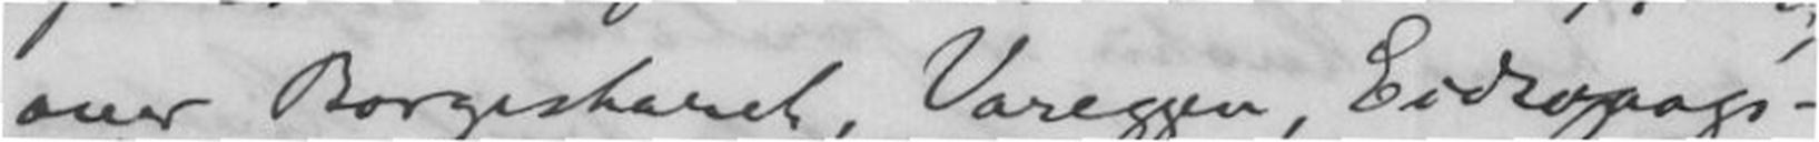over Borgeskaret, Vareggen, Eidsvaags-
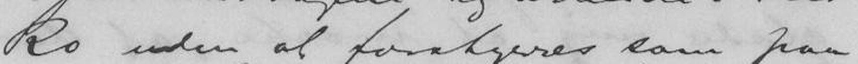og Ro uden at forstyrres som paa
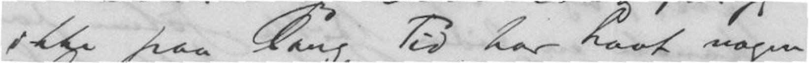ikke paa Lang Tid har havt nogen
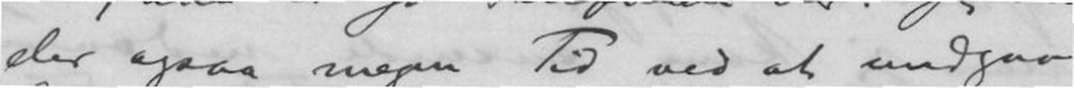der ogsaa megen Tid ved at undgaa
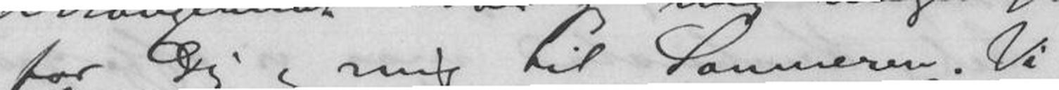for Dig og mig til Sommeren. Vi
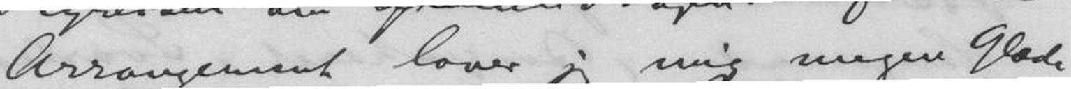Arrangement lover jeg mig megen Glæde
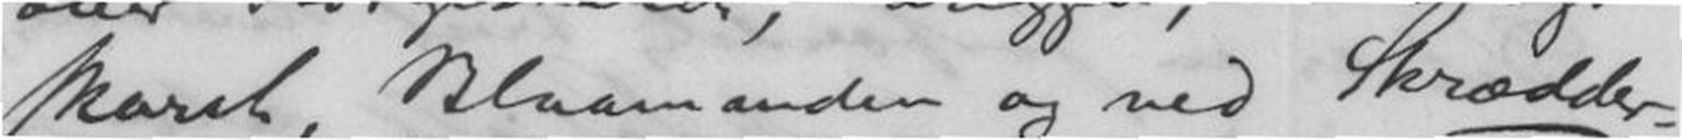skaret, Blaamanden og ned Skrædder-
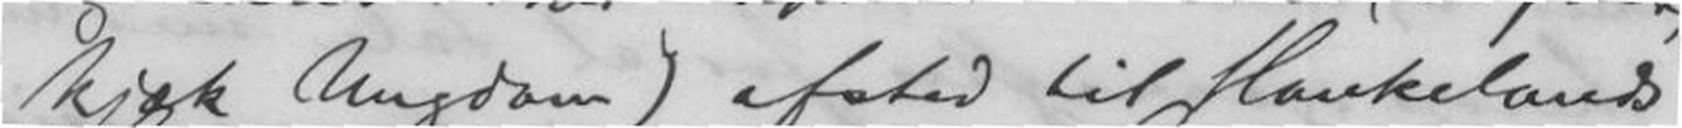kjæk Ungdom) afsted til Haukelands
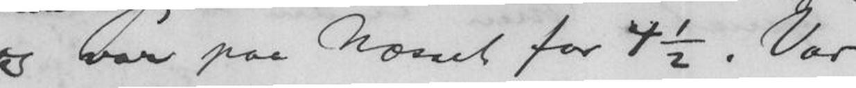og var paa Næsset for 4 ½. Var
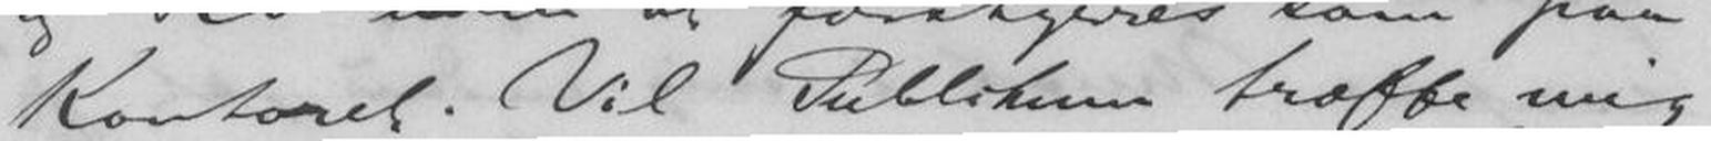Kontoret. Vil Publikum træffe mig
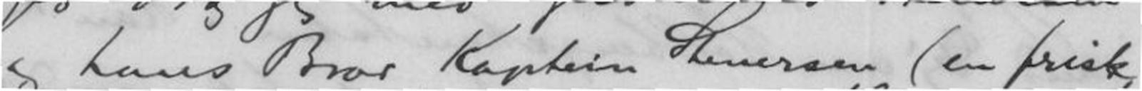og hans Bror Kaptein Stenersen (en frisk,
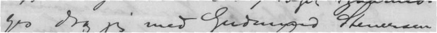ges drog jeg med Gudmund Stenersen
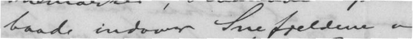baade indover Snefjeldene og
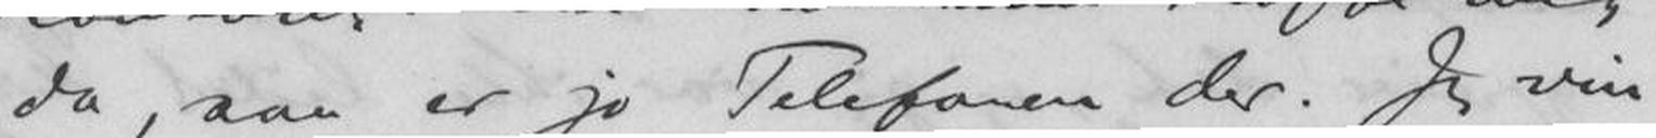da, saa er jo Telefonen der. Jeg vin-
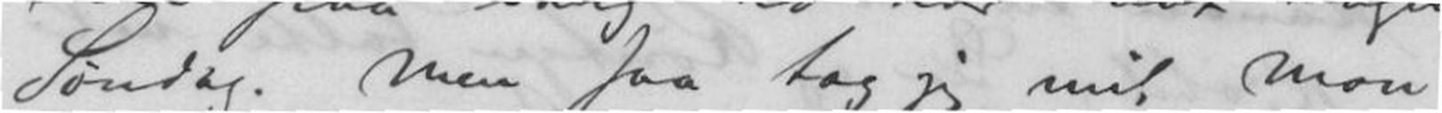Søndag. Men saa tog jeg mit Mon
